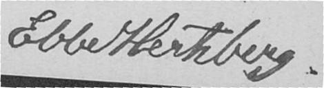Ebbe Hertzberg.
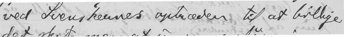ved Svenskernes optræden til at billige
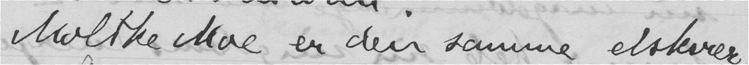Moltke Moe er den samme elskvær-
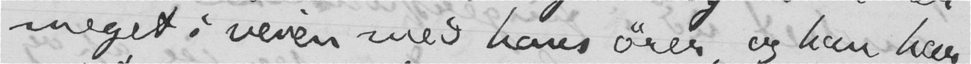meget i veien med hans ører, og han har
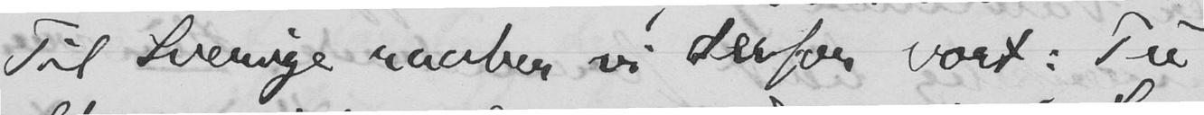Til Sverige raaber vi derfor vort: Tu
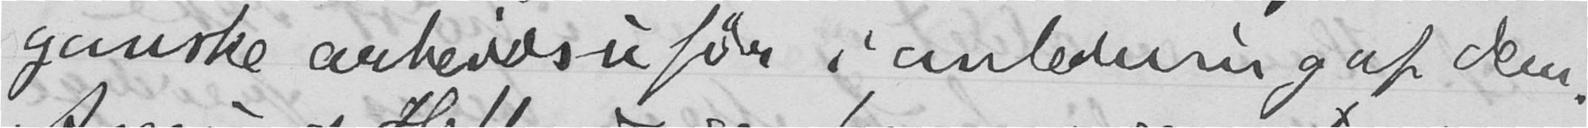ganske arbeidsufør i anledning af dem.
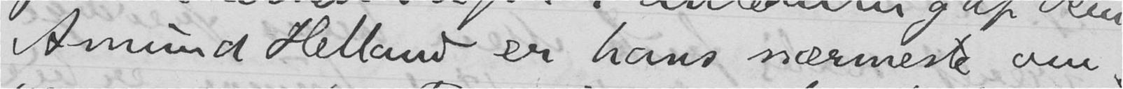Amund Helland er hans nærmeste om-
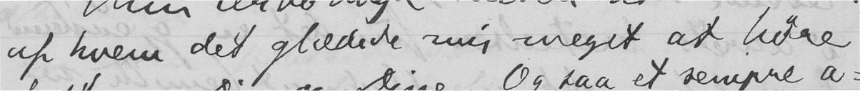af hvem det glædede mig meget at høre
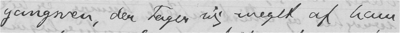gangsven, der tager sig meget af ham.
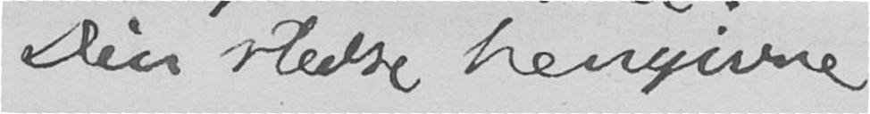Din stedse hengivne
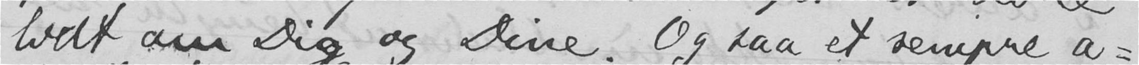lidt om Dig og Dine. Og saa et sempre a-
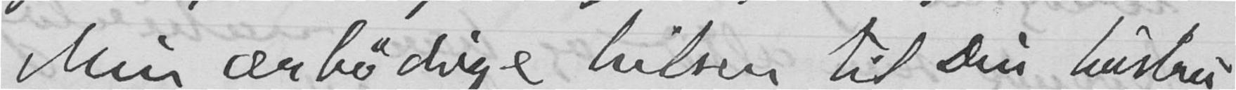Min ærbødige hilsen til Din hustru,
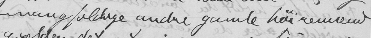mangfoldige andre gamle høiremænd
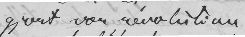gjort vor revolution.
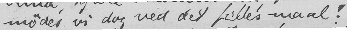mødes vi dog ved det fælles maal!
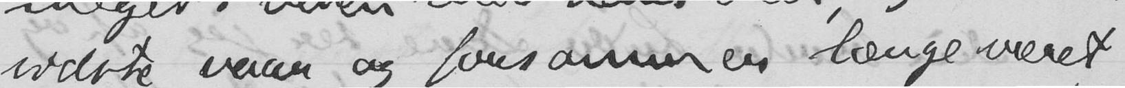sidste vaar og forsommer længe været
